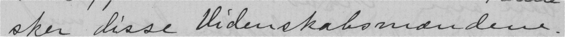sker disse videnskabsmændene.
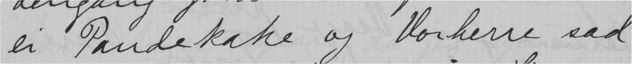ei Pandekake og Vorherre sad
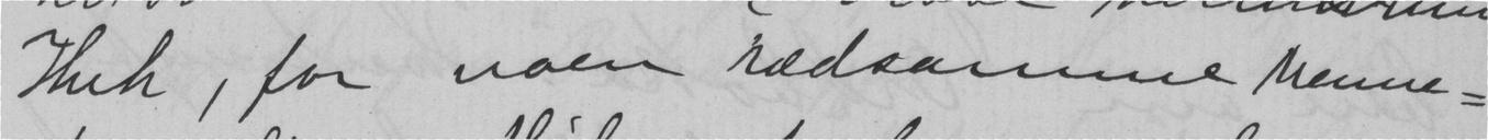Huk, for noen rædsamme menne-
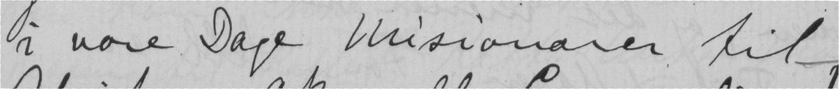i vore Dage misionærer til
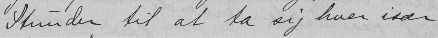Stunder til at ta sig hver især
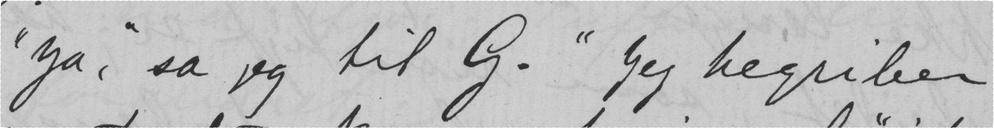"Ja," sa jeg til G. "Jeg begriber
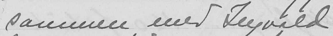sammen med Ingvald
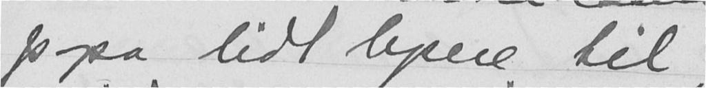papa lidt lysere til
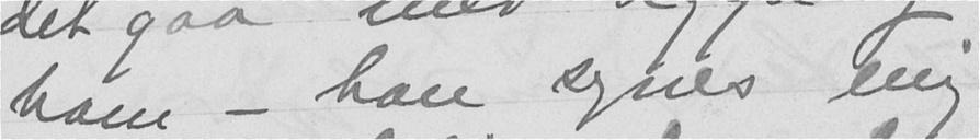ham - han synes mig
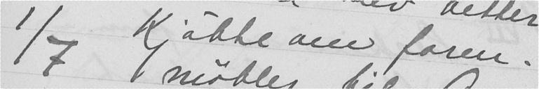1/7 Kjøbte om form.
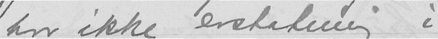har ikke erstatning i
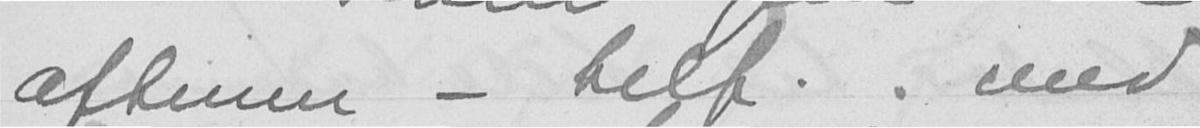aftenen - telf. med
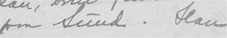paa Sund. Han
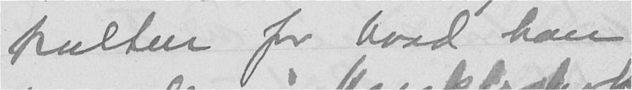trulten for hvad han
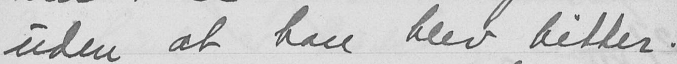uden at han blev bitter.
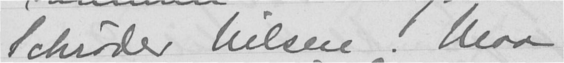Schrøder Nilsen. Maa
